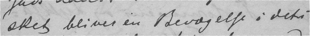sket bliver en Bevægelse i dets
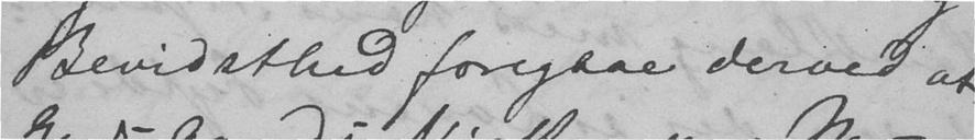Bevidsthed foregaae derved at
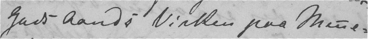Guds Aands Virken paa Menne-
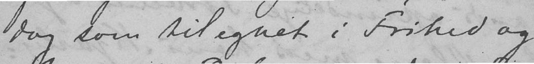dog som tilegnet i Frihed og
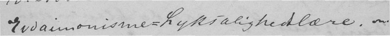Eudaimonisme-Lyksalighedslære.
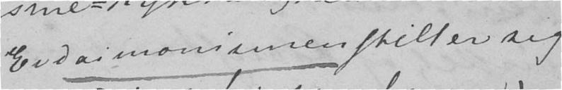Eudaimonismen stiller sig
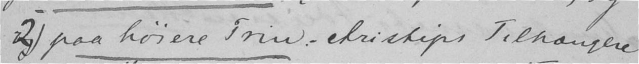2) paa høiere Trin. Aristips Tilhængere
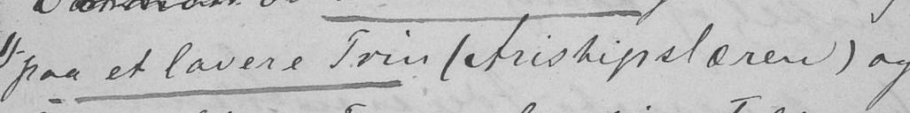1) paa et lavere Trin (Aristipslæren) og
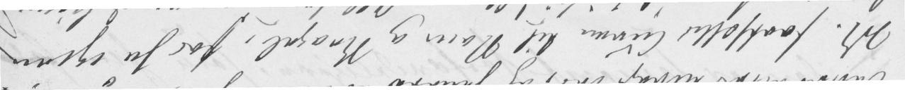M. fortsætter Turen til Rom og Neapel, for så igjen
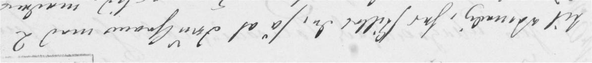til Venedig, her skilles de, så at Fru Gram med 2
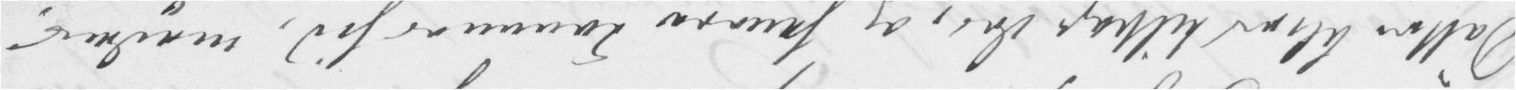Døttre bliver tilbage der, og senere kommer hid, medens
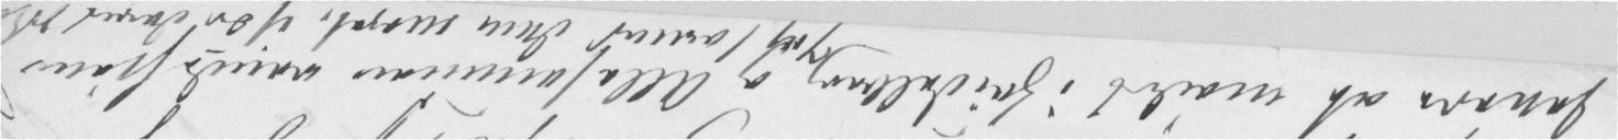senere at mødes i Heidelberg og Allesammen vende hjem
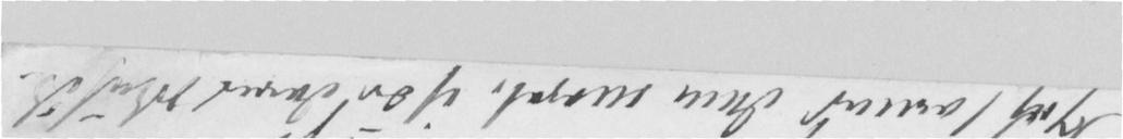Jeg savner dem meget, især deres Musik
